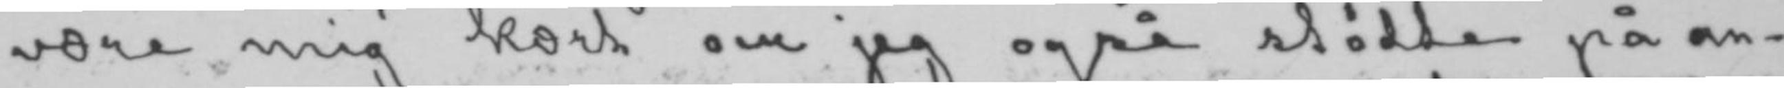være mig kært om jeg også stødte på an-
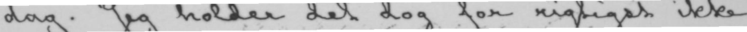dag. Jeg holder det dog for rigtigst ikke
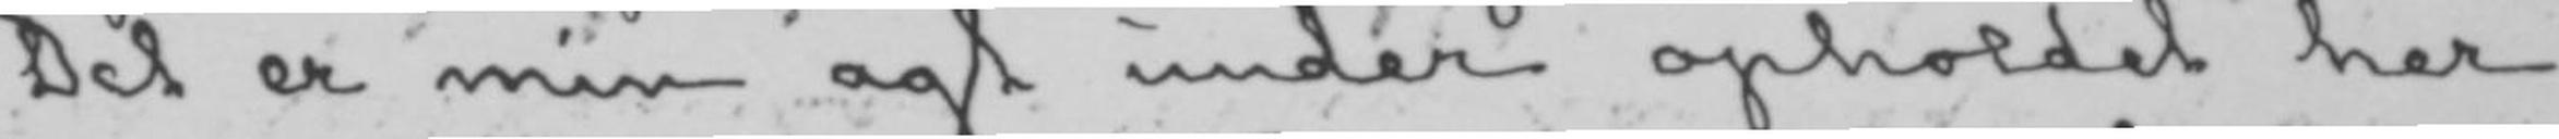Det er min agt under opholdet her
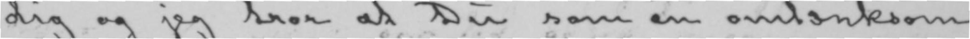dig og jeg tror at Du som en omtænksom
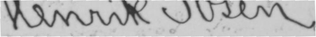Henrik Ibsen.
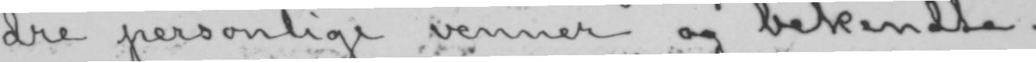dre personlige venner og bekendte.
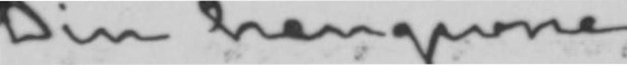Din hengivne
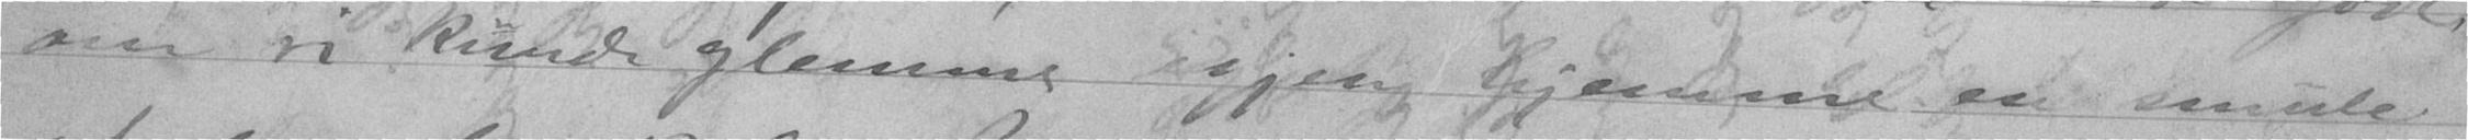om vi kunde glemme igjen hjemme en saante
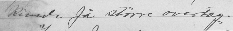kunde få større overtag.
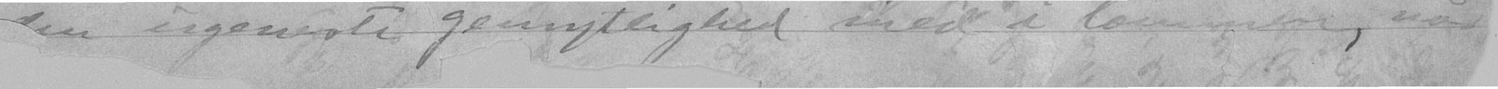den igangsate gemytlighed med i lære, når
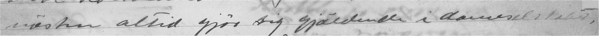nesten altid gjør seg gjældende i dameselskaber,
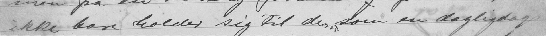ikke bare holder sig til dem som en dagligdags
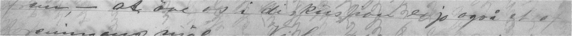frem, - at øve os i diskution er jo også et af
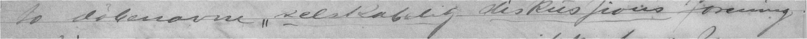to døbsnavne, "selskablig diskustions forening
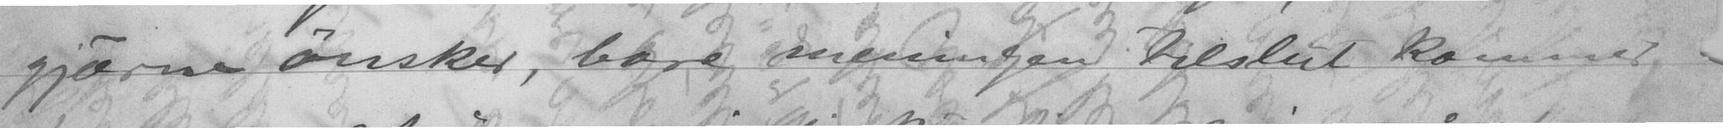gjerne ønsker, bare meningen tilslut kommer
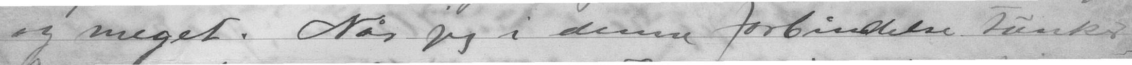og meget. Når jeg i denne forbindelse tenker
